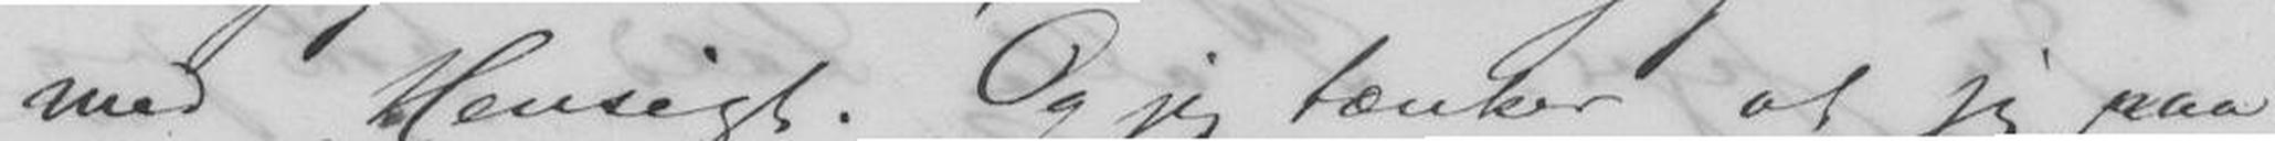med Hensigt. Og jeg tænker at jeg paa
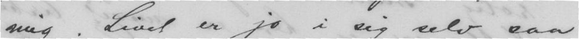mig. Livet er jo i sig selv saa
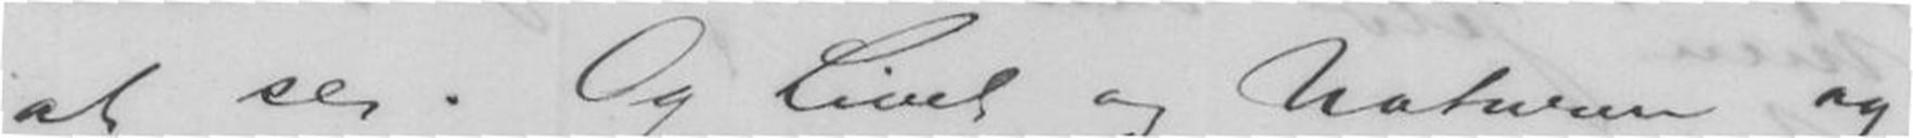at se. Og livet og Naturen og
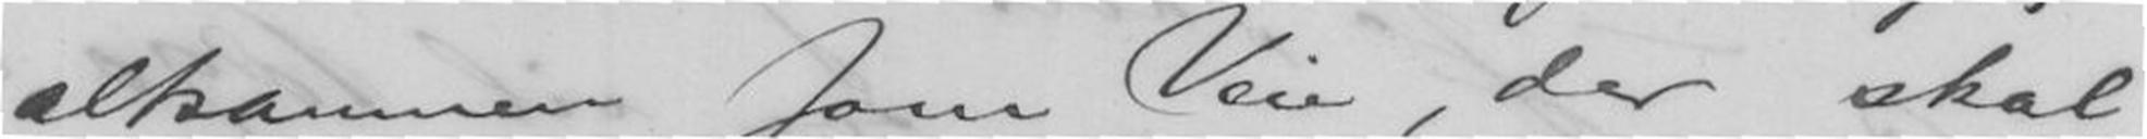altsammen som Veie, der skal
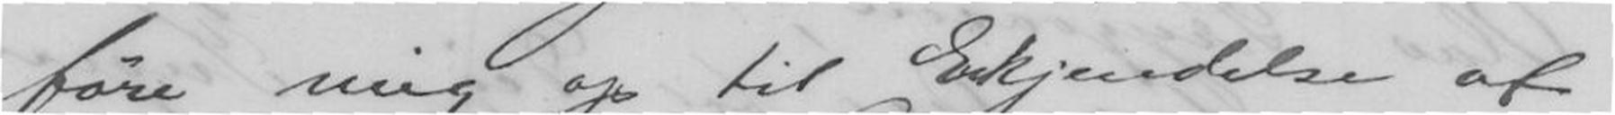føre mig op til Erkjendelse af
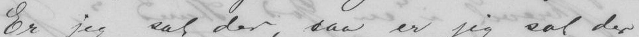Er jeg sat der, saa er jeg sat der
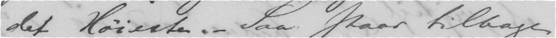det Høieste. - Saa staar tilbage
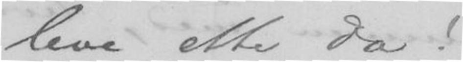leve ette da!"
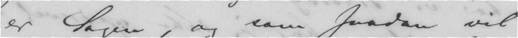er Sagen, og som saadan vil
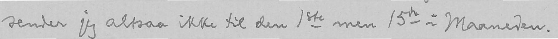sender jeg altsaa ikke til den 1ste men 15de i Maaneden.
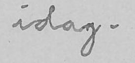idag.
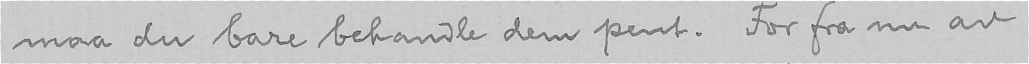maa du bare behandle dem pent. For fra nu av
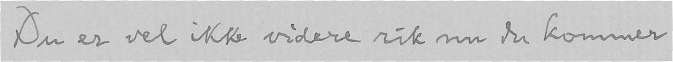Du er vel ikke videre rik nu du kommer
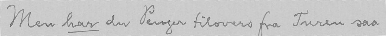Men har du Penger tilovers fra Turen saa
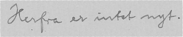Herfra er intet nyt.
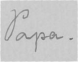Papa.
